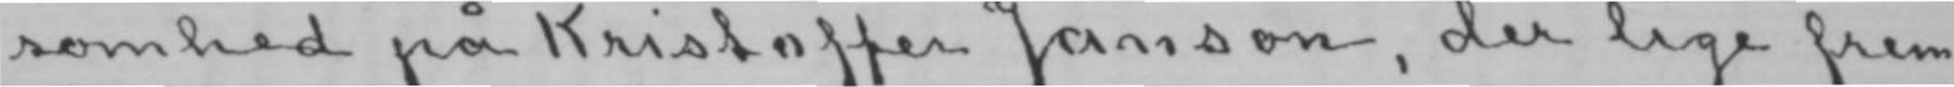somhed på Kristoffer Janson, der lige frem
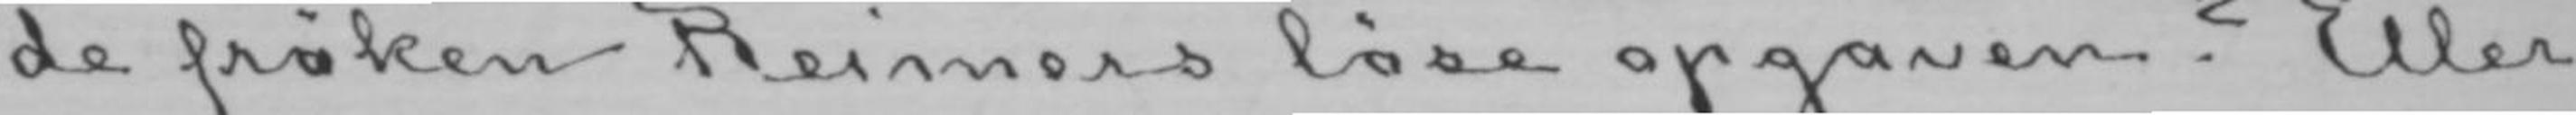de frøken Reimers løse opgaven? Eller
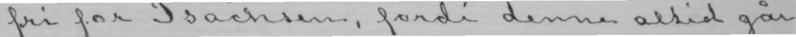fri for Isachsen, fordi denne altid går
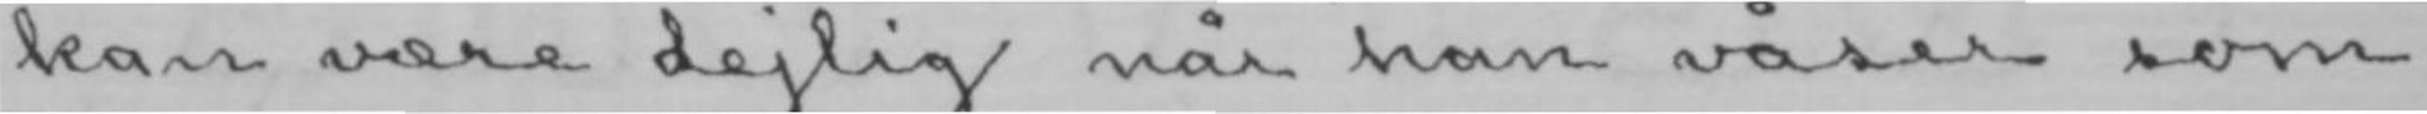kan være dejlig når han våser som
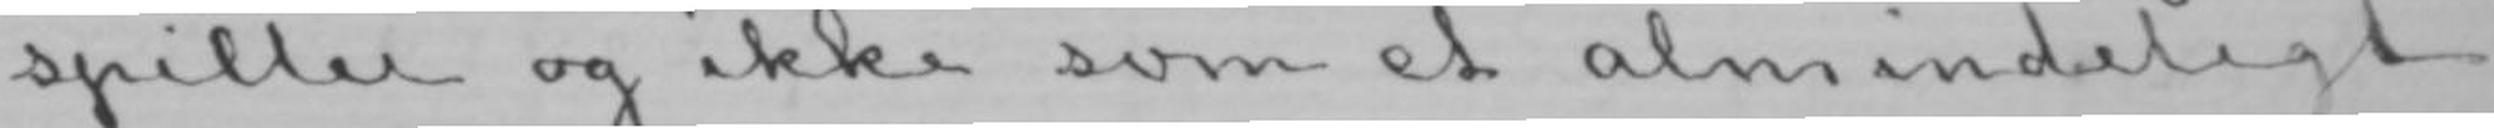spiller og ikke som et almindeligt
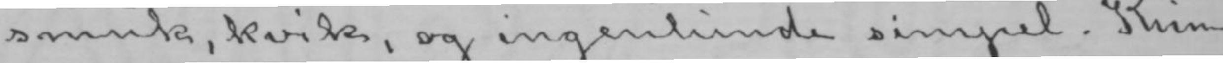smuk, kvik, og ingenlunde simpel. Kun-
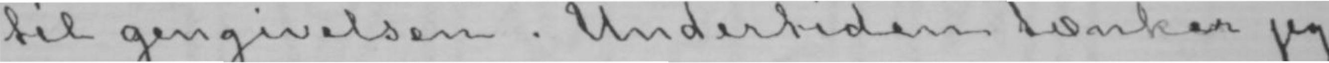til gengivelsen. Undertiden tænker jeg
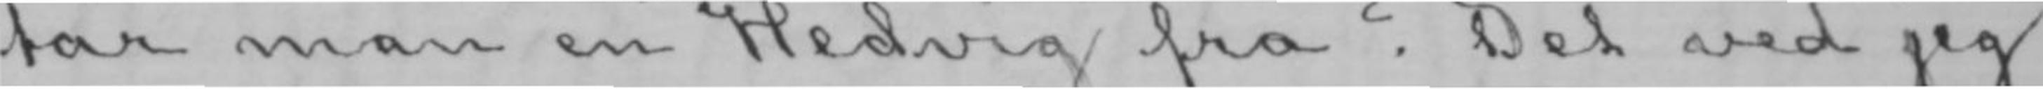tar man en Hedvig fra? Det ved jeg
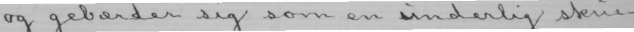og gebærder sig som en sunderlig skue-
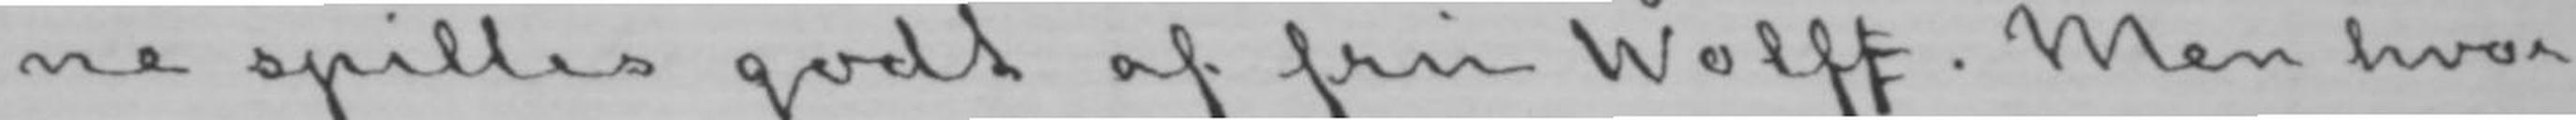ne spilles godt af fru Wolff. Men hvor
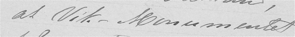at Vik-Monumentet
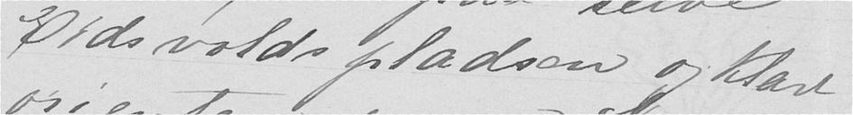Eidsvoldspladsen og klart
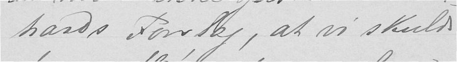hards Forslag, at vi skulde
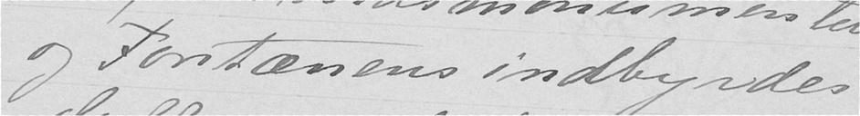og Fontænens indbyrdes
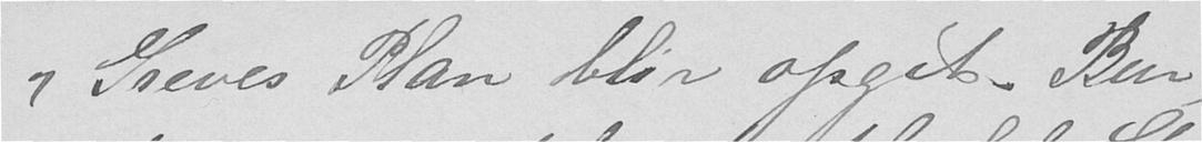Greves Plan blir opgit. Bur-
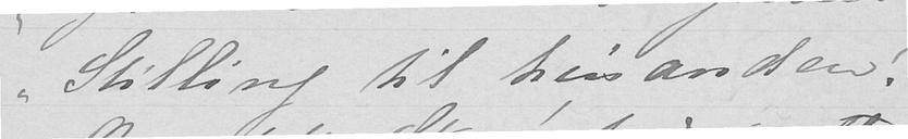Stilling til hinanden?
